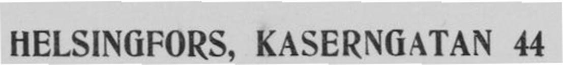HELSINGFORS, KASAERNGATAN 44
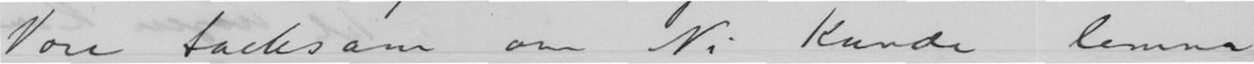Vore tacksam om Ni kunde lemna
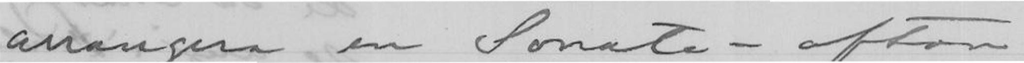arrangera en Sonate - afton
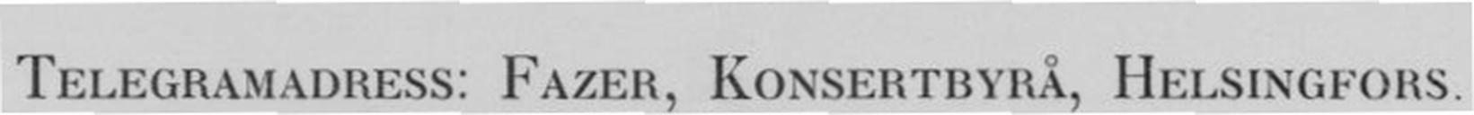TELEGRAMADRESS: FAZER, KONSERTBYRÅ, HELSINGFORS.
